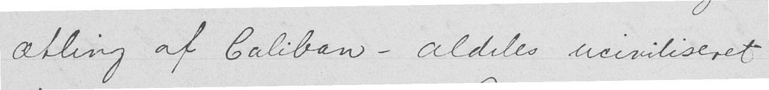ætling af Caliban — aldeles uciviliseret
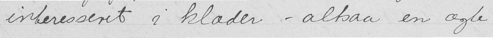interesseret i klæder — altsaa en ægte
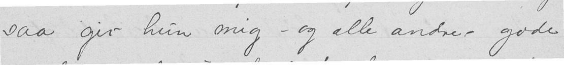saa gir hun mig — og alle andre — gode
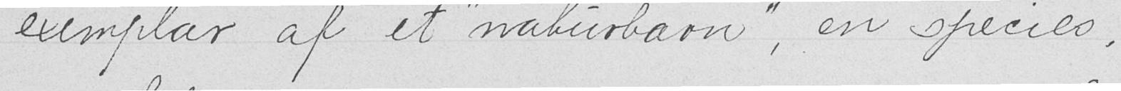exemplar af et "naturbarn", en species,
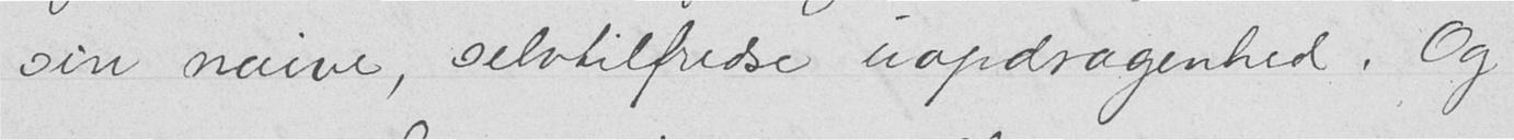sin naive, selvtilfredse uopdragenhed. Og
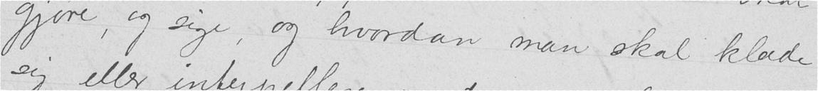gjøre, og sige, og hvordan man skal klæde
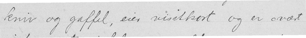kniv og gaffel, eier visitkort og er svært
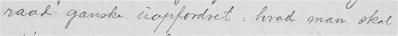raad ganske uopfordret: hvad man skal
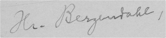Hr. Bergendahl,
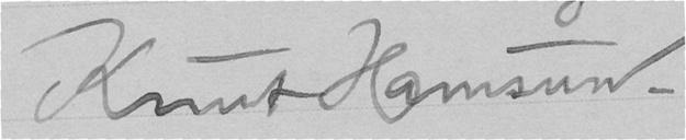Knut Hamsun.
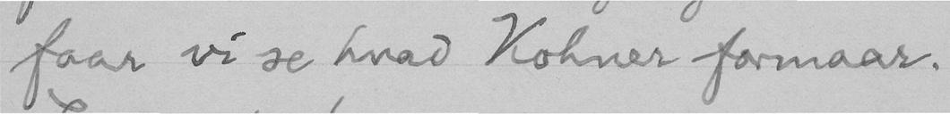faar vi se hvad Kohner formaar.
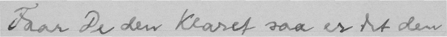Faar De den klaret saa er det den
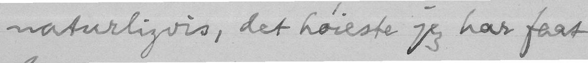naturligvis, det høieste jeg har faat
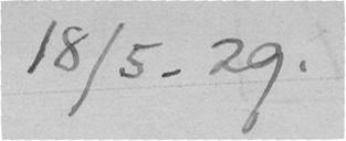18/5.29.
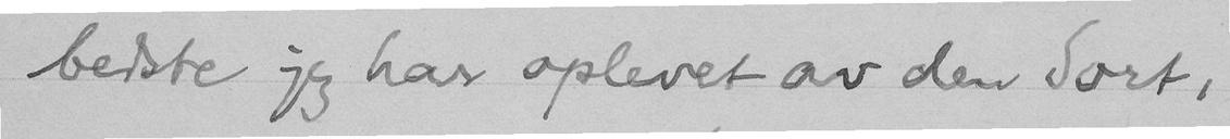bedste jeg har oplevet av den Sort,
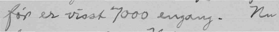før er visst 7000 engang. Nu
